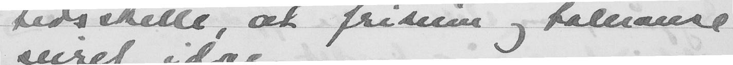tidsskille, at frisinn og toleranse
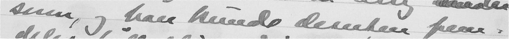sum, og han kunde desuten frem-
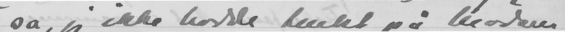sa, jeg ikke hadde tenkt på Madsen
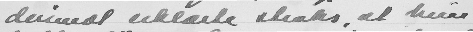derimot erklærte straks, at hun
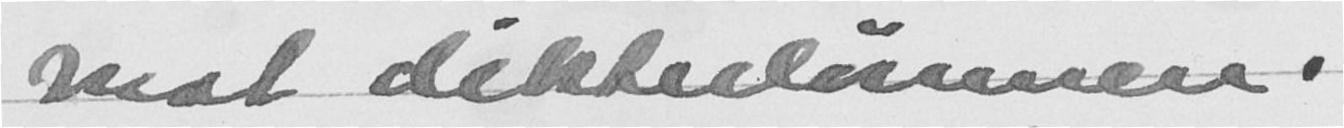mot dikterlønnen.
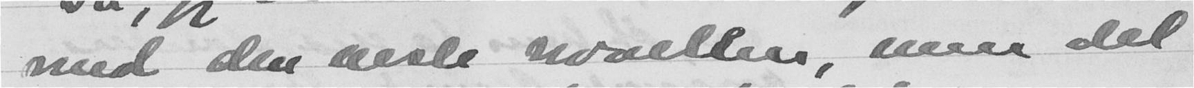med den neste novellen, men det
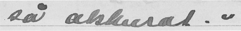så akkurat."
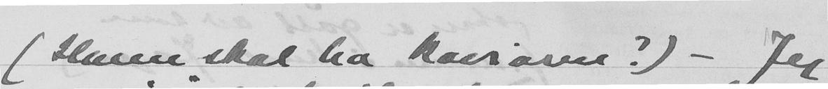(Henni skal ha kaviare?) - Jeg
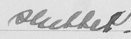sluttet.
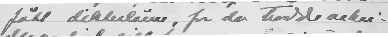fått dikterlønn, for da hadde arbei-
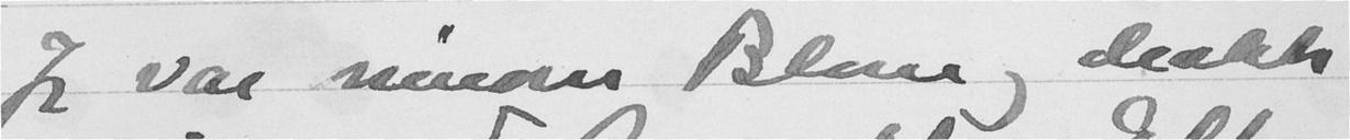Jeg var innom Blom og drakk
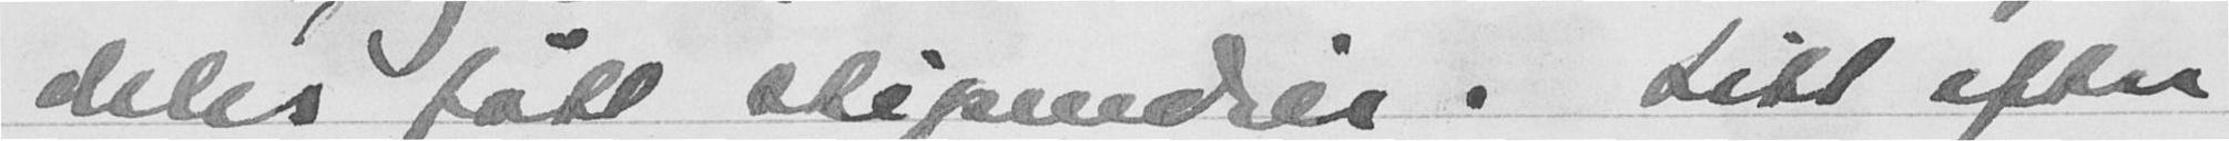deles fått stipendier. Litt efter
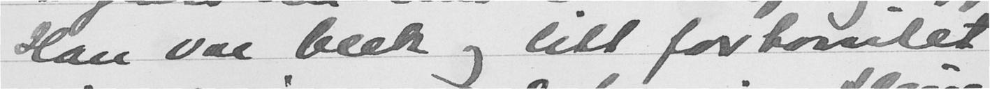Han var blek og litt fortumlet -
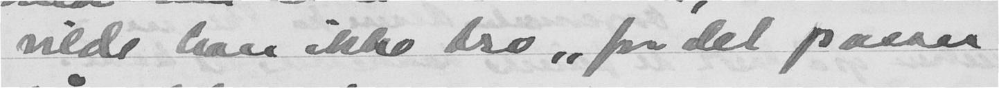vilde han ikke tro, "for det passer
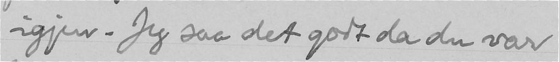igjen. Jeg saa det godt da du var
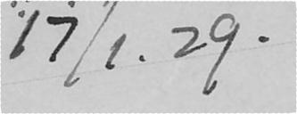17/1. 29.
